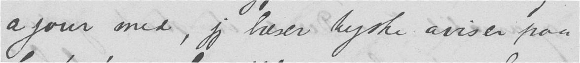a jour med, jeg leser tyske aviser paa
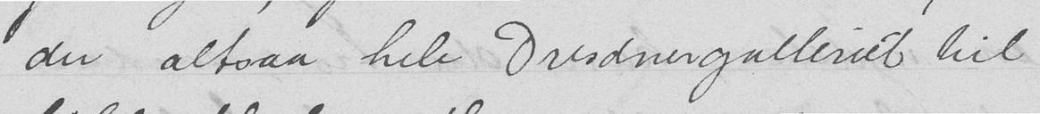du altsaa hele Dresdengalleret til
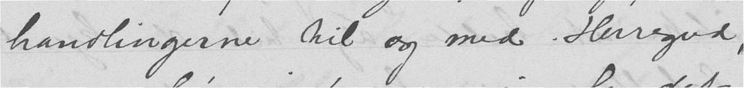handlingerne til og med. Herregud,
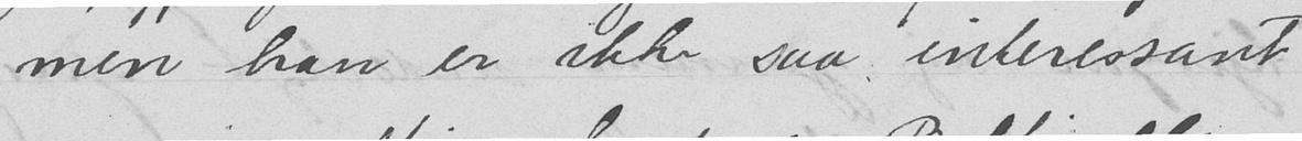men han er ikke saa interessant
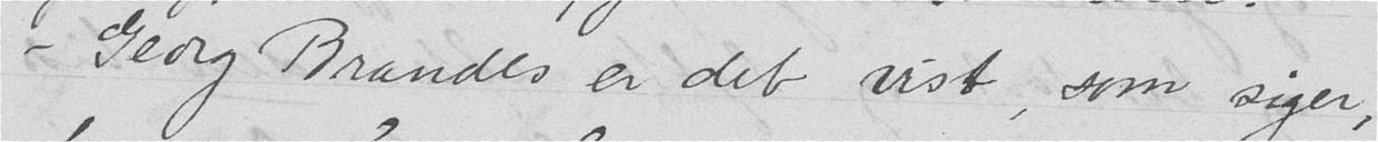- Georg Brandes er det vist, som siger,
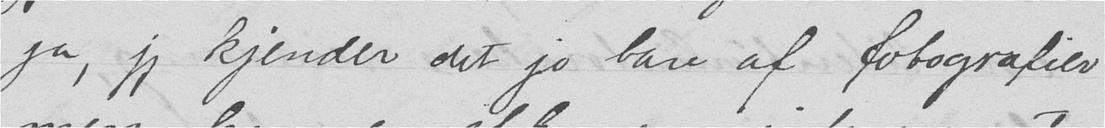ja, jeg kjender det jo bare af fotografier
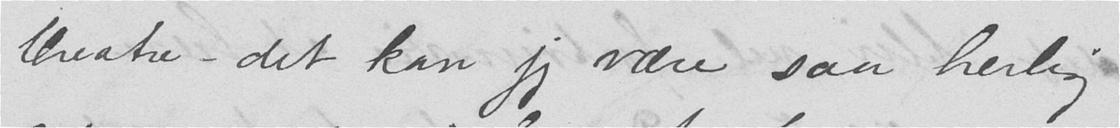theatre - det kan jeg være saa herlig
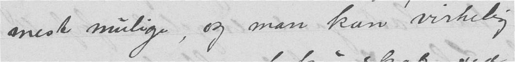mest mulige, og man kan virkelig
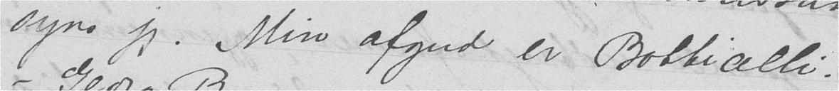syns jeg. Min afgud er Botticelli.
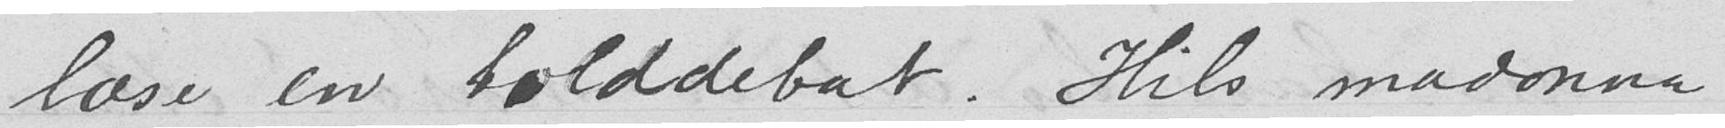læse en tolddebat. Hils madonna
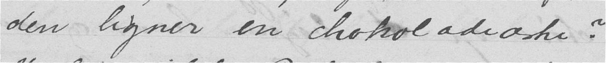den ligner en chokoladeæske?
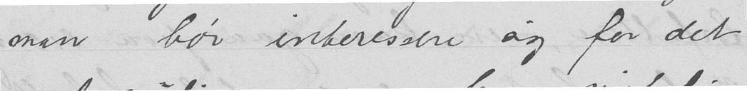man bør interessere sig for det
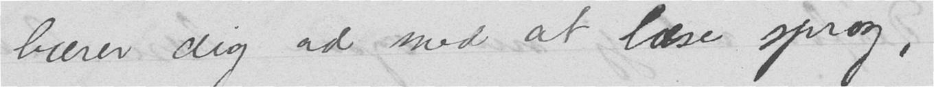bærer dig ad med at læse sprog,
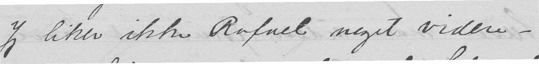Jeg liker ikke Rafael noget videre -
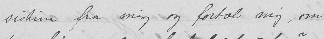sistina fra mig og fortæl mig, om
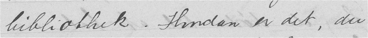bibliothek. Hvordan er det, du
